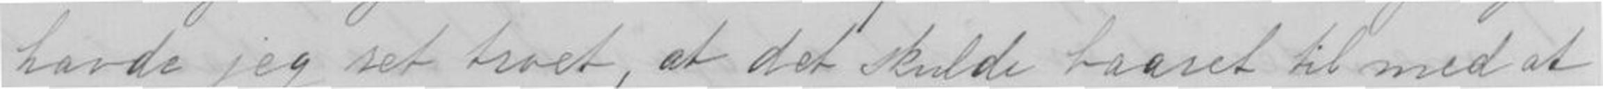havde jeg ret troet, at det skulde baaret til med at
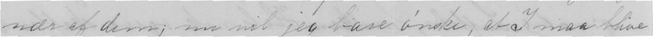nær af dem, no vil jeg bare ønske, at I maa blive
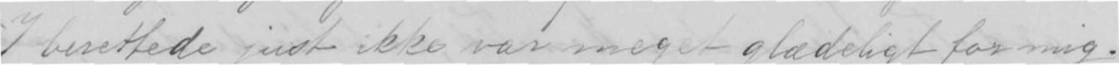I berettede just ikke var meget glædeligt for mig.
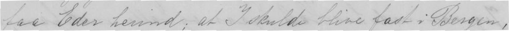faa Eder heimd; at I skulde blive fast i Bergen,
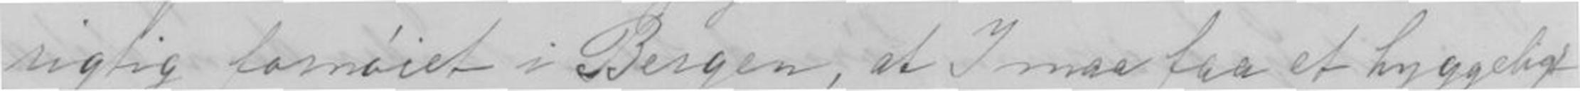rigtig lomøiet i Bergen, at I maa faa et hyggelig
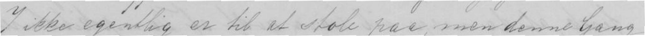I ikke egentlig er til at stole paa, men denne Gang
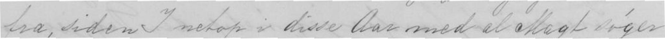fra, siden I netop i disse Aar med al Magt søger
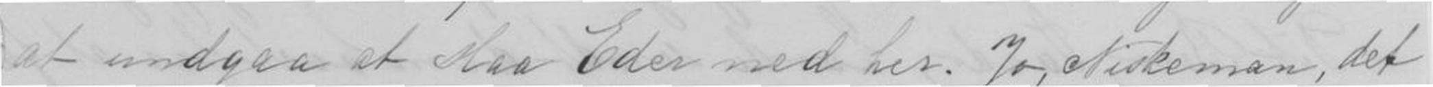at ungaa at Saa Eder med her. Jo, Niskeman, det
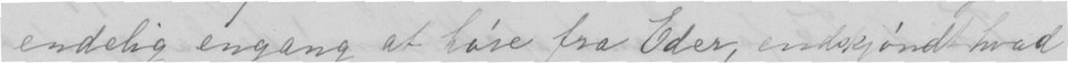endelig engang at høre fra Eder, endskjøndt hvad
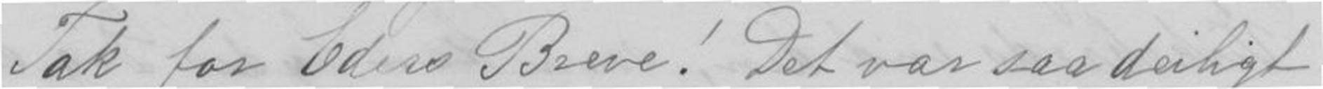Tak for Edens Breve! Det var saa deiligt
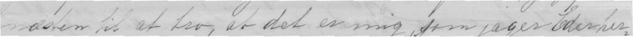næsten til at tro, at det er mig, som jager Edern her-
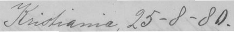Kristiania,25-8-80
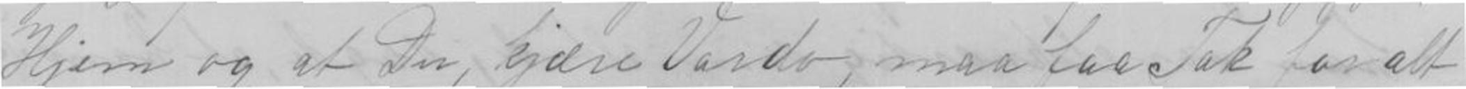Hjem og Din, Kjære Vando, maa faa Tak for alt
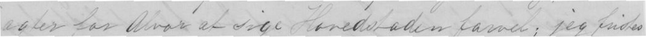agter for alvor at sige Hovedstaden farvel; jeg fristes
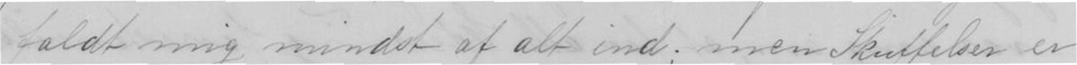faldt mig mindst af alt ind, men Skuffelser er
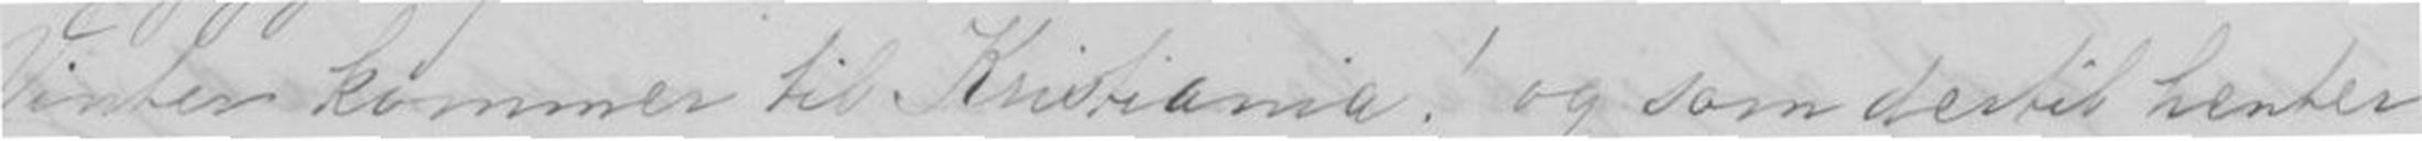Vinter kommer til Kristiania! og som dertil henter
